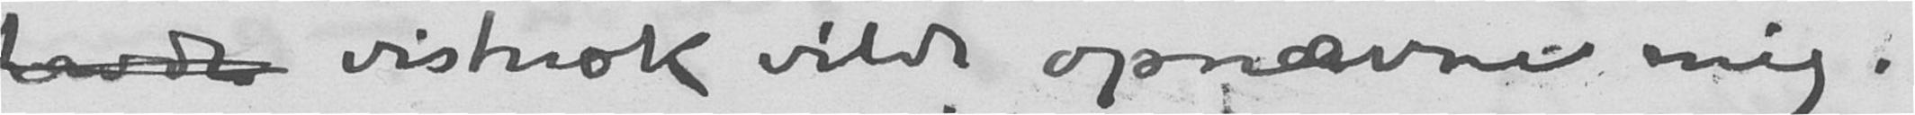havde vistnok vilde opnævne mig.
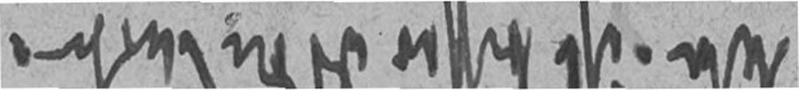Ihre ist besser als die Andere
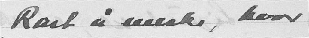Rart å smake, hvor
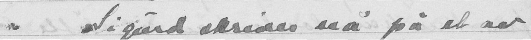Sigurd skriver nå på et av
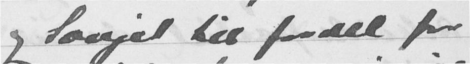og Sovjet til fordel for
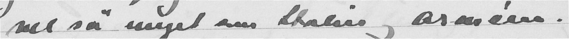vel så meget som Stalin og Armcen.
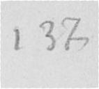137
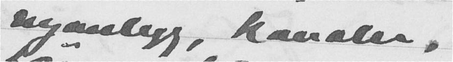nyanlegg, kanaler,
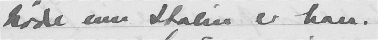hode enn Stalin er han.
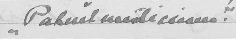"Patentundscsen."
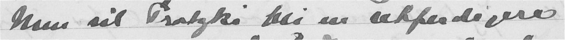Men vil Trotsjki bli en rettferdigere
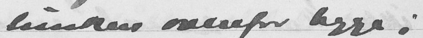lunken ovenfor begge,
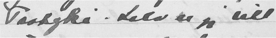Trotsjki. Selv er jeg litt
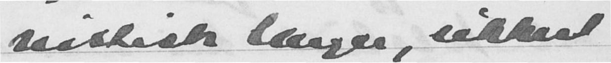nistisk lenger, sikkert
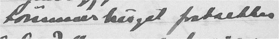Lommerhuset fortsetter
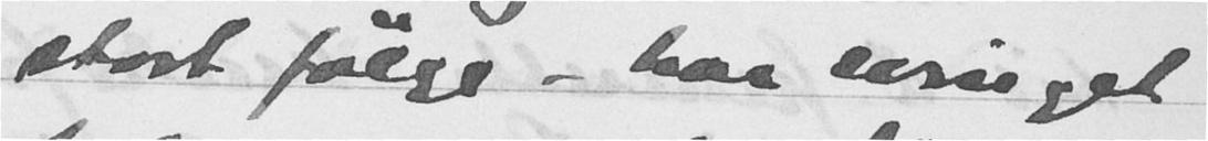stort følge - har svinget
